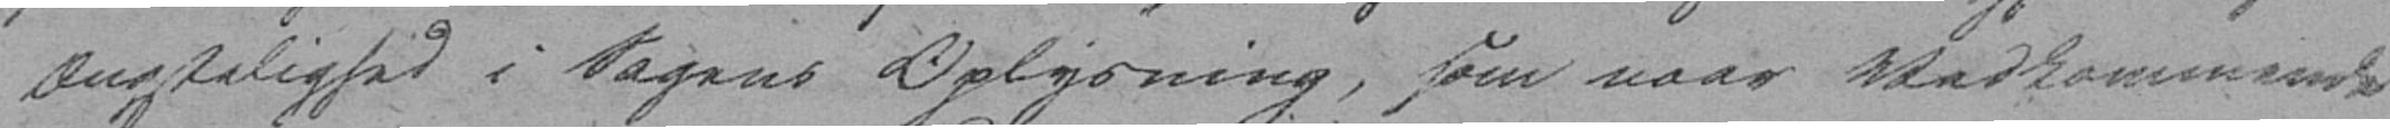Ængstelighed i Sagens Oplysning, som naar Vedkommende
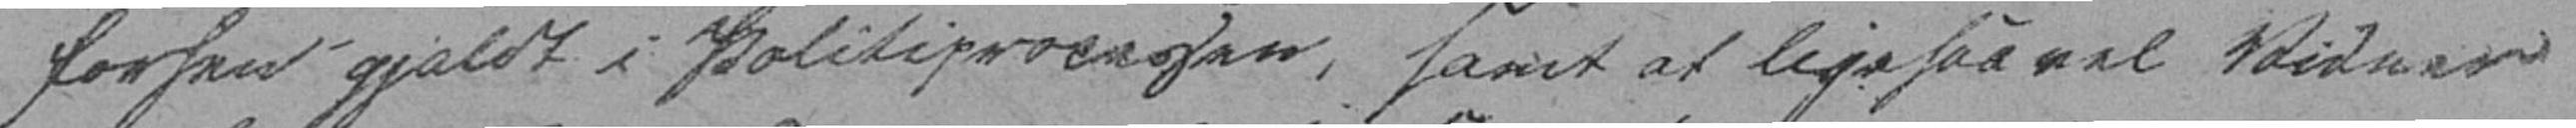forhen gjaldt i Politiprocessen, samt at ligesaa vel Vidner
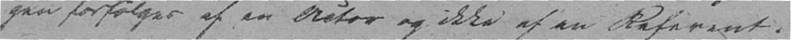gen forfølges af en Actor og ikke af en Referent.
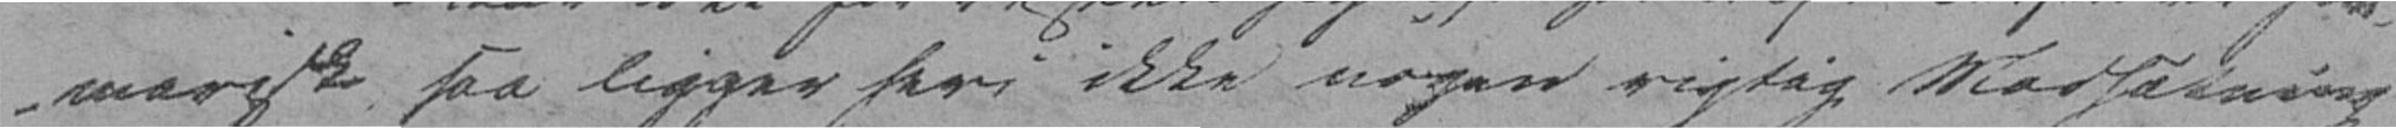marisk, saa ligger heri ikke nogen rigtig Modsætning
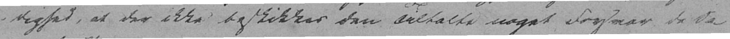dighed, at der ikke beskikkes den Tiltalte noget Forsvar da Sa-
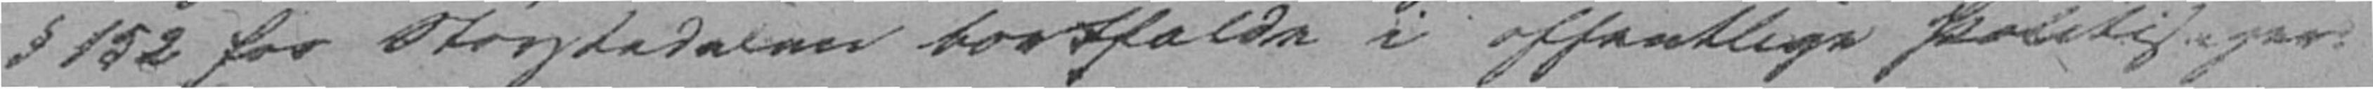§ 152 for Størstedelen bortfalde i offentlige Politisager.
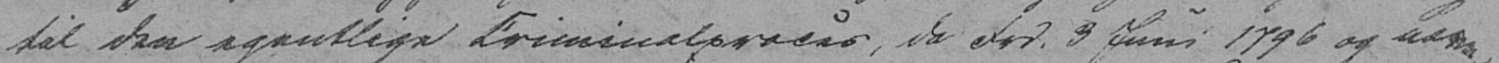til den egentlige Criminalproces, da Frd. 3 Juni 1796 og nær-
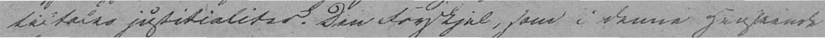tiltales justitialiter. Den Forskjel, som i denne Henseende
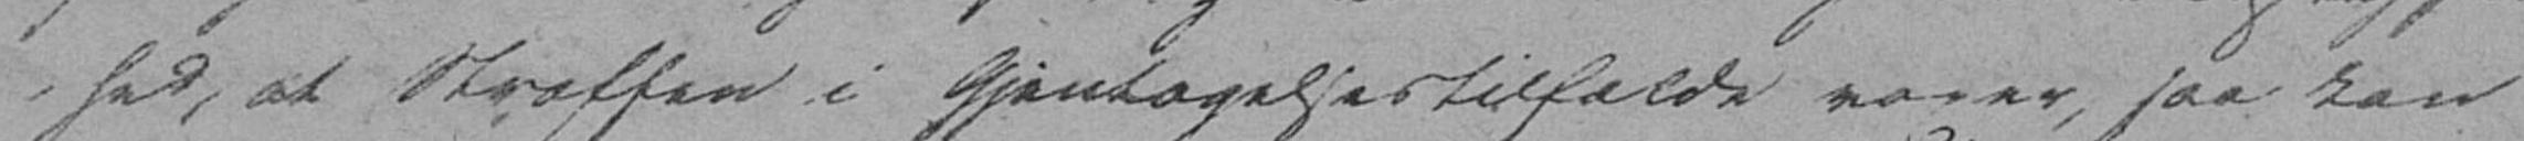hed, at Straffen i Gjentagelsestilfælde varer, saa kan
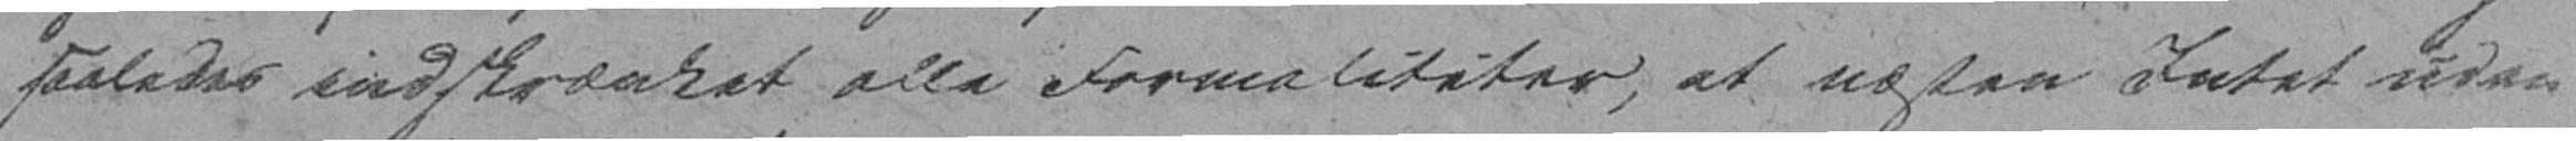saaledes indskrænket alle Formaliteter, at næsten Intet uden
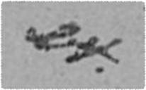24.
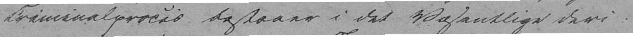Criminalproces bestaaer i det Væsentlige deri.
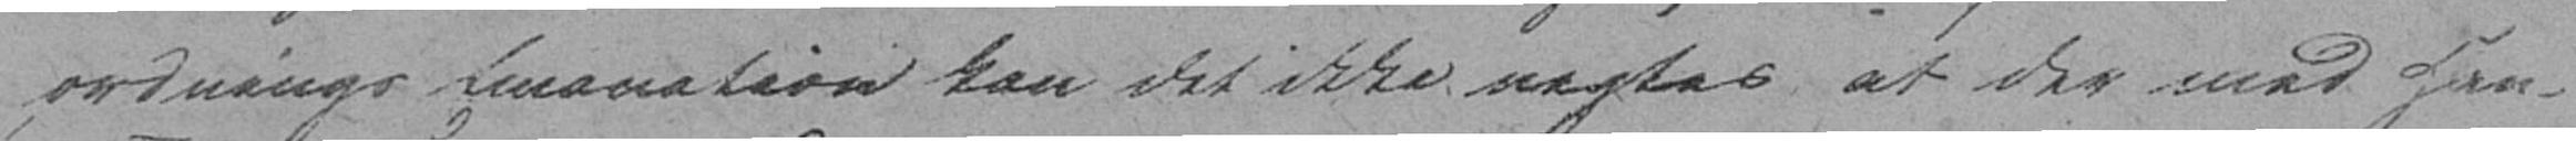ordnings Imanation kan det ikke nægtes at der med Hen-
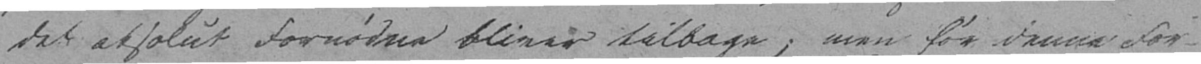det absolut Fornødne bliver tilbage; men før denne For-
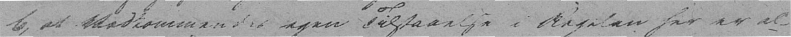6, at Vedkommendes egen Tilstaaelse i Regelen her er al-
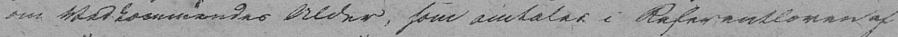om Vedkommendes Alder, som omtales i Referentloven af
